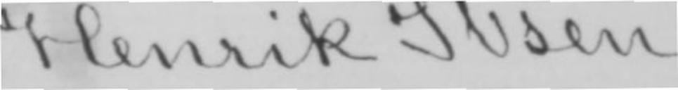Henrik Ibsen.
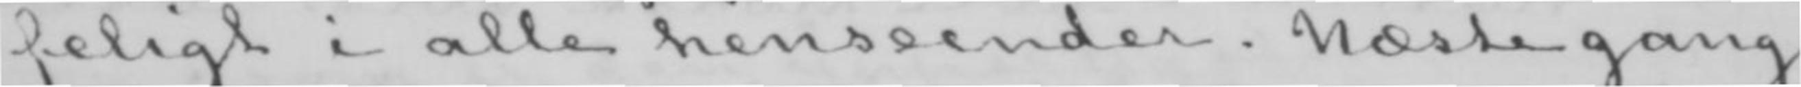feligt i alle henseender. Næste gang
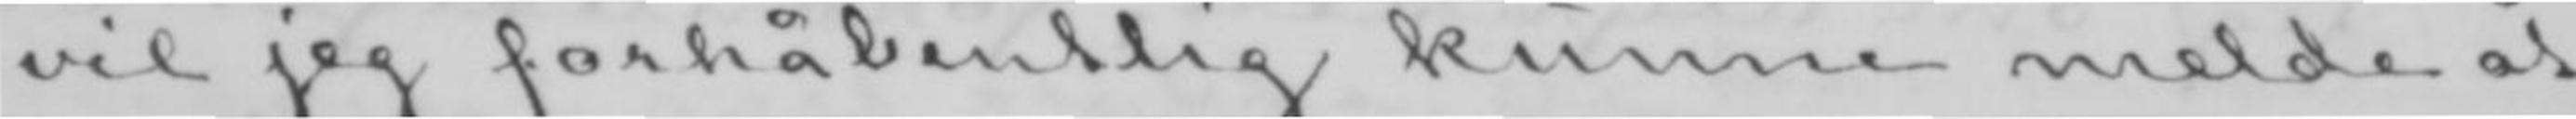vil jeg forhåbentlig kunne melde at
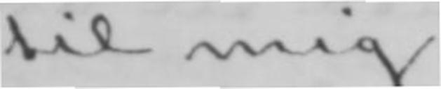til mig.
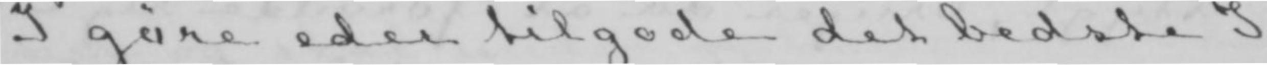I gøre eder tilgode det bedste I
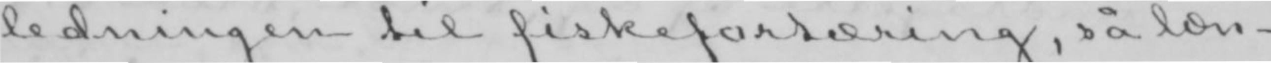ledningen til fiskefortæring, så læn-
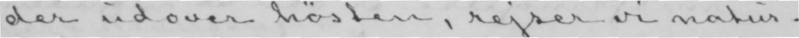der udover høsten, rejser vi natur-
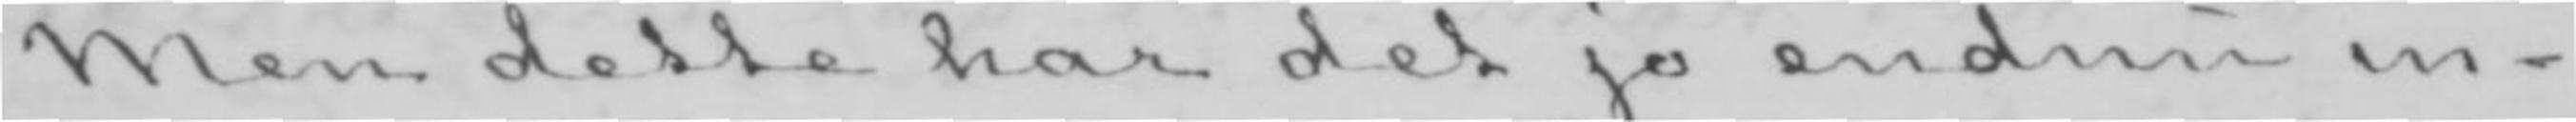Men dette har det jo endnu in-
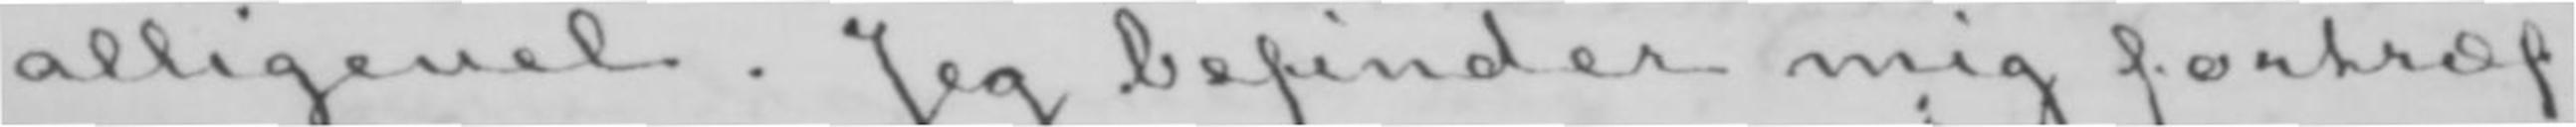alligevel. Jeg befinder mig fortræf-
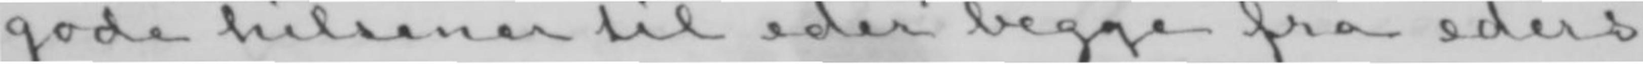gode hilsener til eder begge fra eders
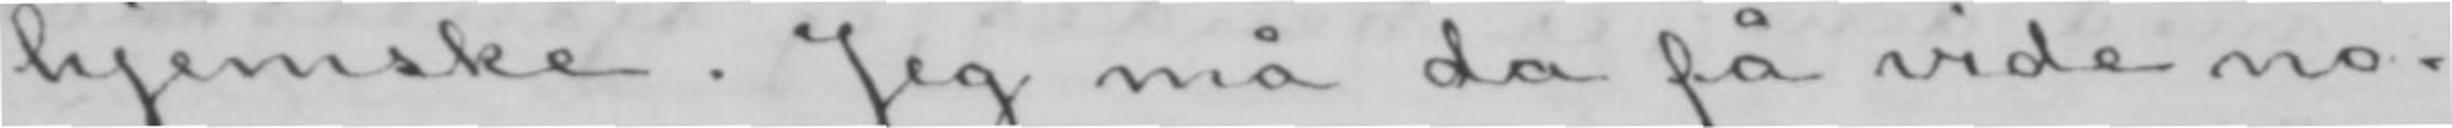hjemske. Jeg må da få vide no-
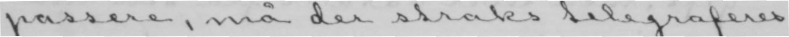passere, må der straks telegraferes
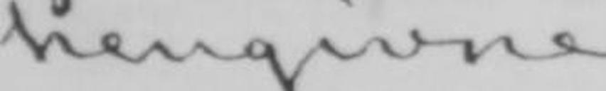hengivne
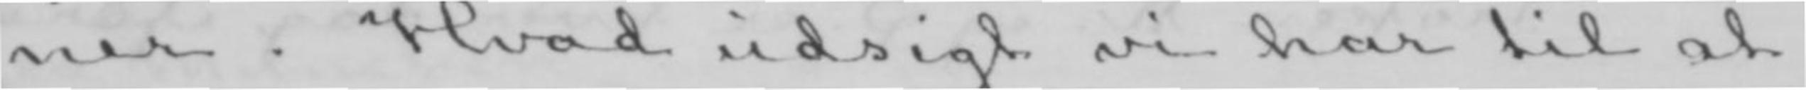ner. Hvad udsigt vi har til at
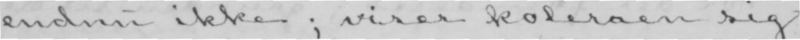endnu ikke; viser koleraen sig
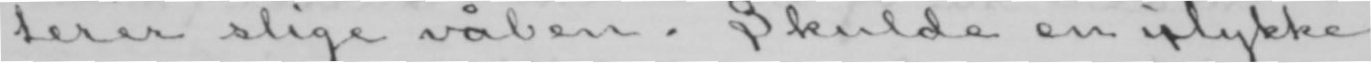terer slige våben. Skulde en ulykke
